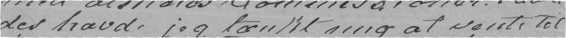des havde jeg tænkt mig at vente til
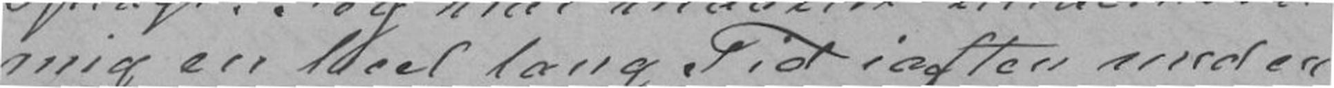mig en heel lang Tid iaften med en
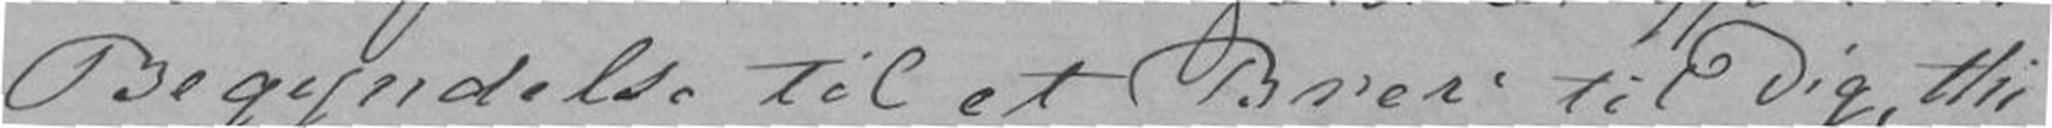Begyndelse til et Brev til Dig, thi
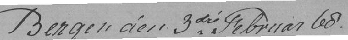Bergen den 3die Februar 68
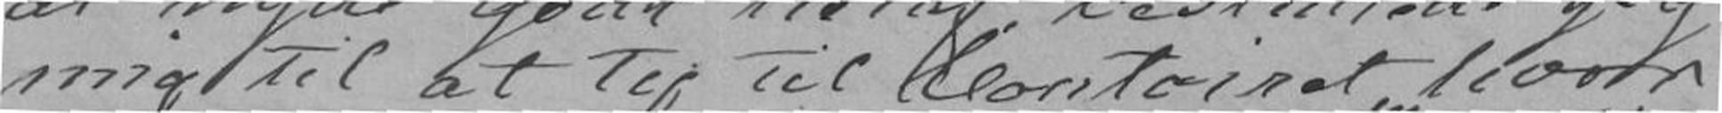mig til at ty til Contoiret hvor
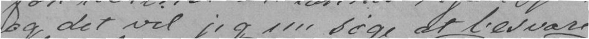og det vil jeg nu søge at besvare
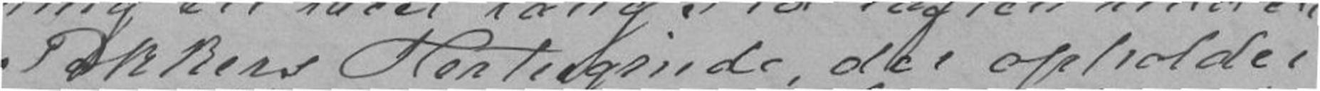Pokkers Hertuginde, der opholder
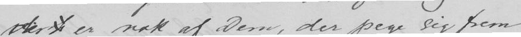er nok af Dem, der pege sig frem
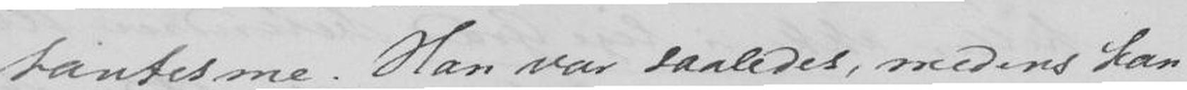tantisme. Han var saaledes, medens han
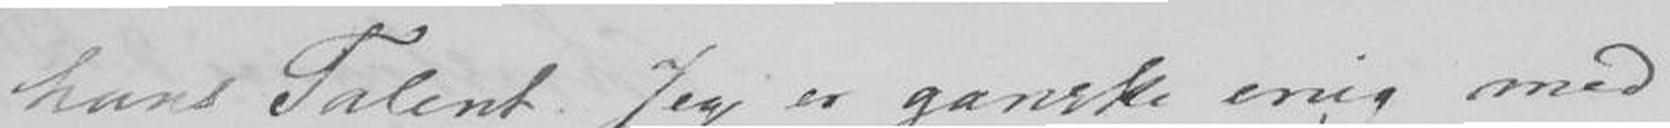hans Talent. Jeg er ganske enig med
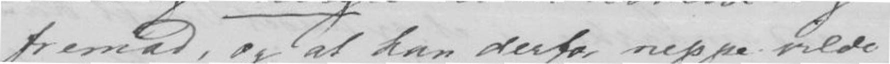fremad, og at han derfor neppe vilde
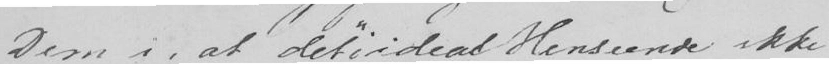Dem i, at det i den Henseende ikke
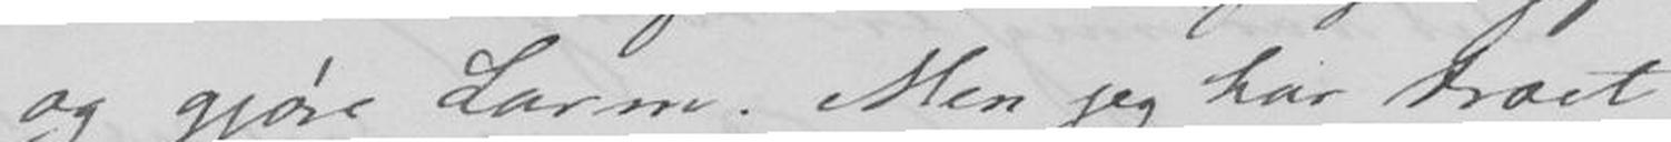og gjøre Larm. Men jeg har troet
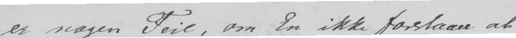er nogen Feil, om En ikke forstaar at
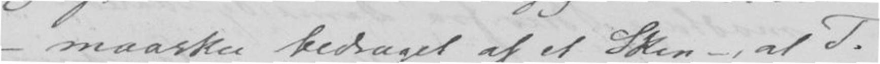- maaskee bedraget af et Skin -, at T.
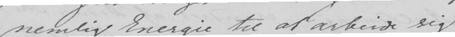nemlig Energie til at arbeide sig
